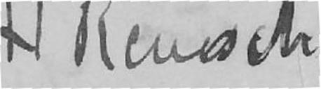A Reusch
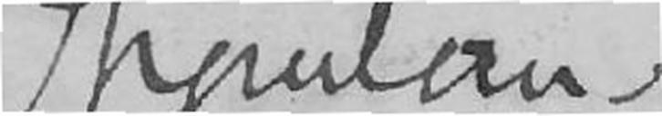Thaulow
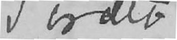Jordet
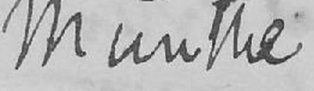Munthe
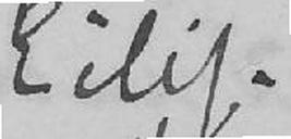Eilif.
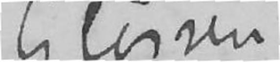Glørsen
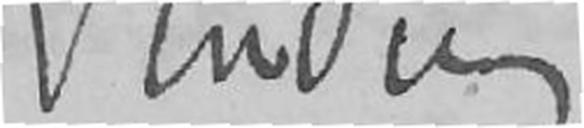Sinding
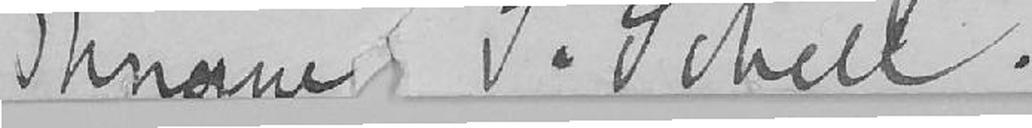Thrane S. Scheel
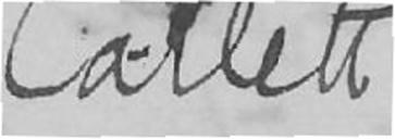Collett
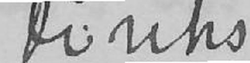Diriks
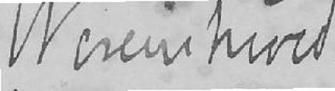Werenskiold
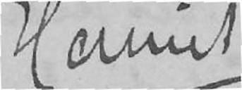Harriet
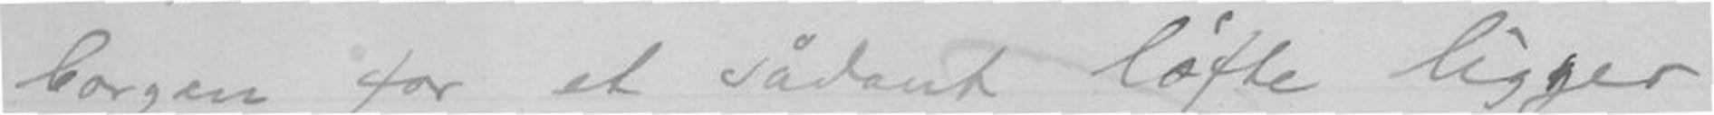borgen for et sådant løfte ligger
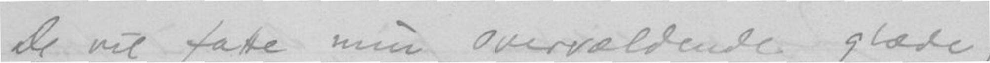De vil fatte min overvældende glæde,
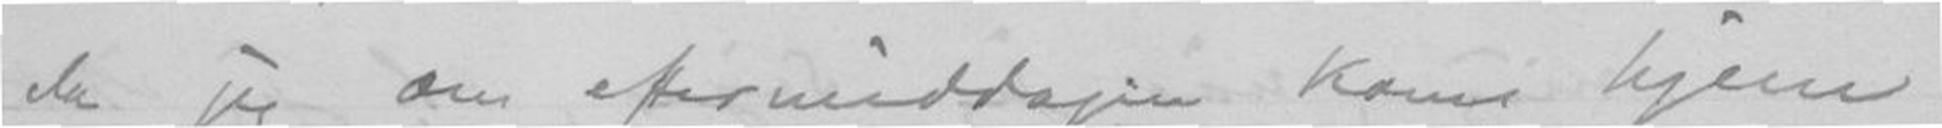da jeg om eftermiddagen kom hjem
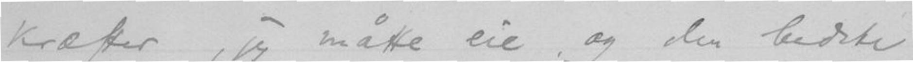kræfter, jeg måtte eie og den bedste
